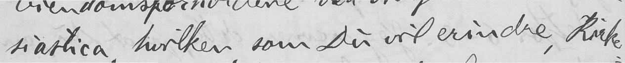siastica, hvilken, som Du vil erindre, Kirke-
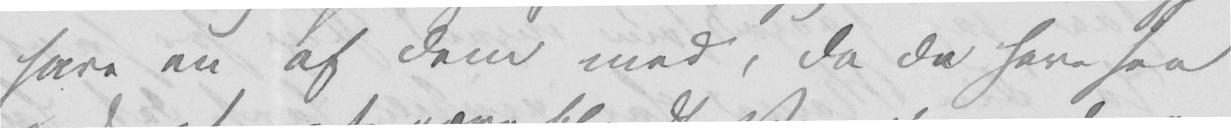have en af dem med, da de have saa
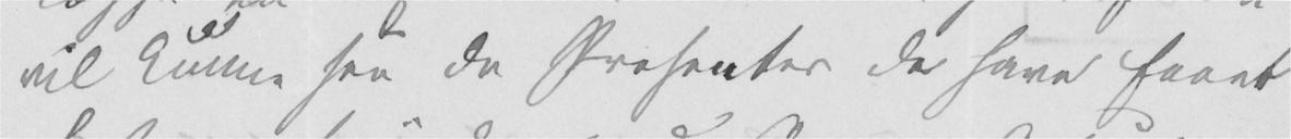vil kunne see de Presenter de have faaet
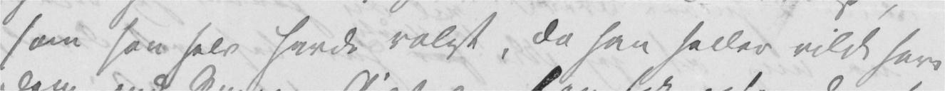som han selv havde valgt, da han heller vilde have
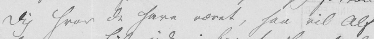Dig hvor de have været, saa vil Alf
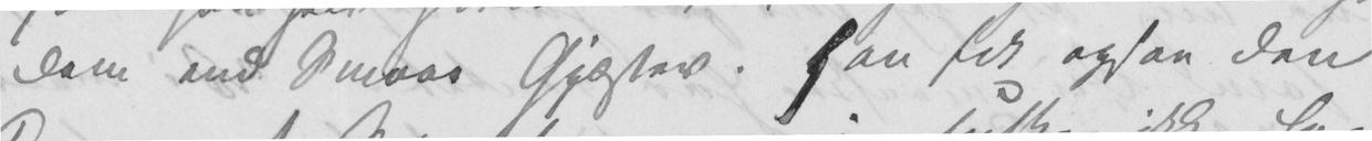dem end Smaae Gjæster. Han fik ogsaa den
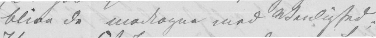blive de modtagne med Venlighed.
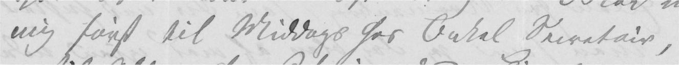mig først til Middags hos Onkel Secretair,
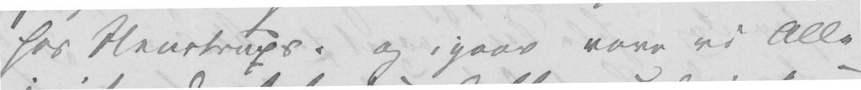hos Stenstrups, og igaar vare vi Alle
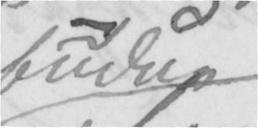budne
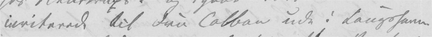inviterede til Fru Colban ude i Kongshavn.
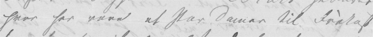hvor her vare et Par Damer til Frokost,
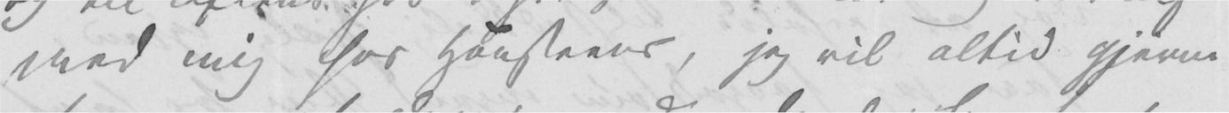med mig hos Hansteens, jeg vil altid gjerne
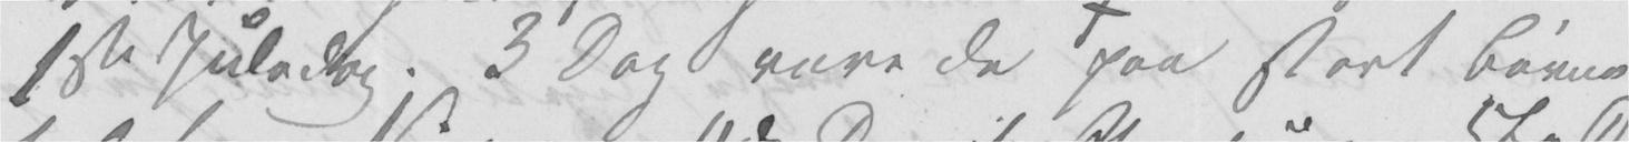1ste Juledag. 3 Dag vare de paa stort Børne-
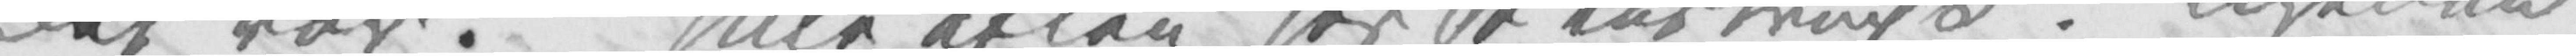det var. Juleaften hos Stenstrups. Ligedan
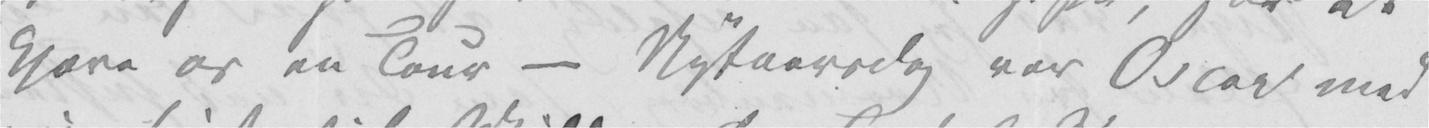kjøre os en Tour – Nytaarsdag var Oscar med
